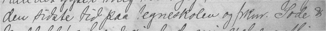den sidste tid paa tegneskolen og frknr. Sode &
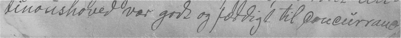tinonshoved var godt og færdigt til concurrance
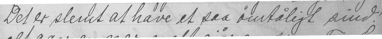Det er slemt at have et saa ømtåligt sind!
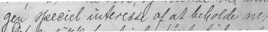gen speciel interesse af at beholde ne
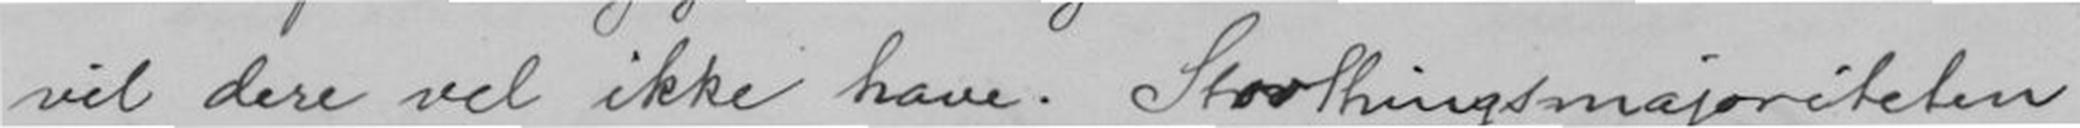vil dere vel ikke have. Storthingsmajoriteten
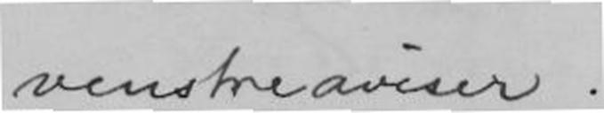venstreaviser.
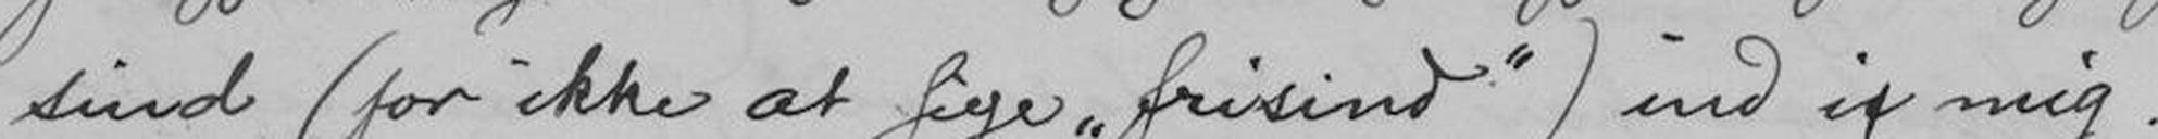sind (for ikke at sige "frisind") ind i mig.
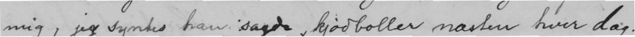mig, jeg synes han sagde kjødboller næsten hver dag.
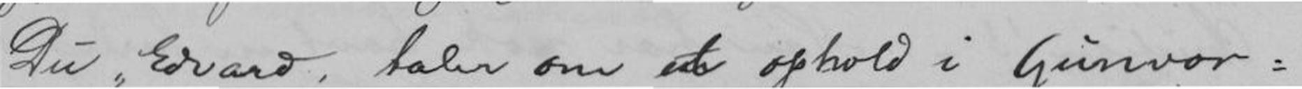Du, Edvard taler om et ophold i Gunvor-
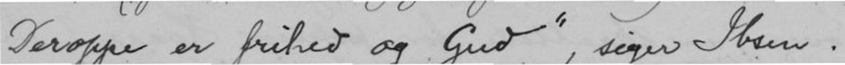Deroppe er frihed og Gud", siger Ibsen.
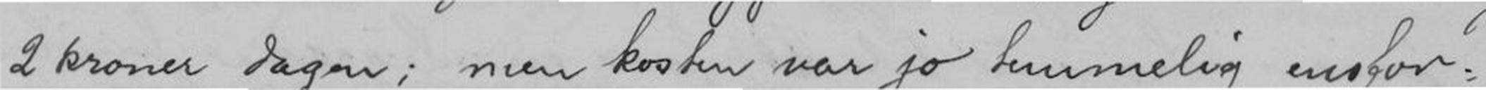- 2 kroner dagen; men kosten var jo temmelig ensfor-
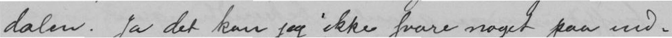dalen. Ja det kan jeg ikke svare noget paa end-
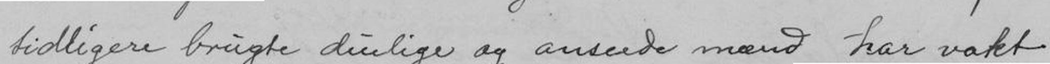tidligere brugte duelige og anseede mænd har vakt
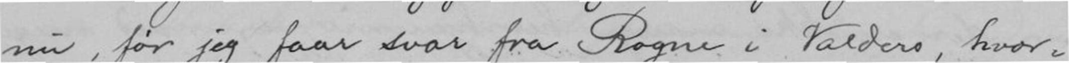nu, før jeg faar svar fra Rogne i Valders, hvor
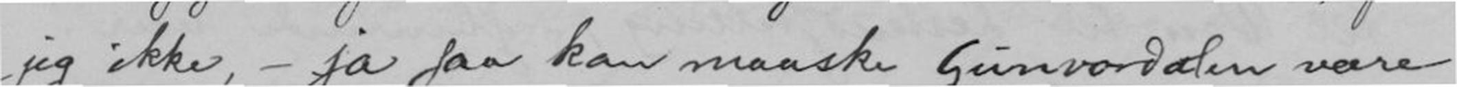jeg ikke, - ja saa kan maaske Gunvordalen være
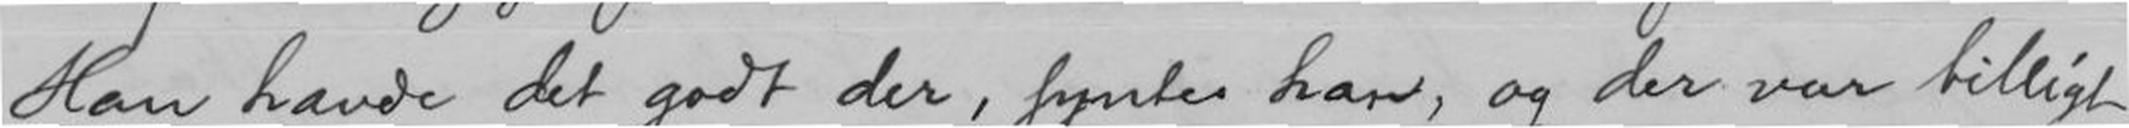Han havde det godt der, synstes han, og der var billigt
